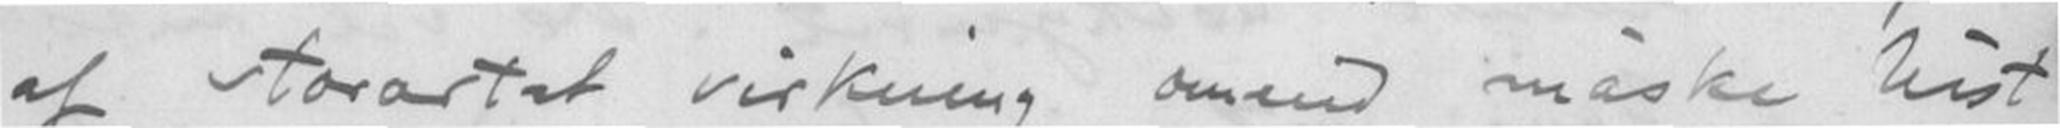af storartet virkning om end måske hist
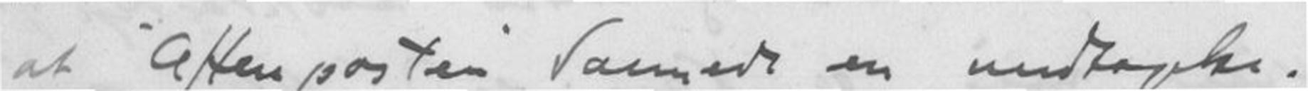at "Aftenposten" Savnede en undtagelse.
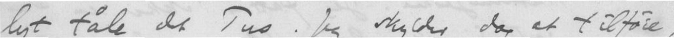lyt tåle det Pus. Jeg skylder dog at tilføie,
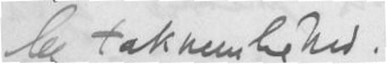be taknemlighed.
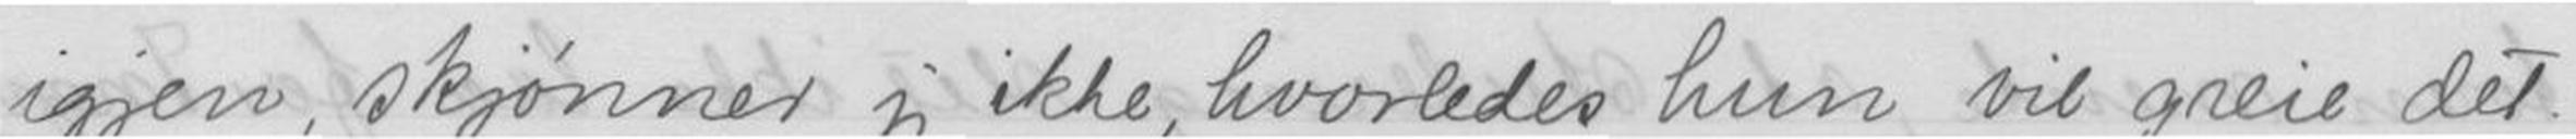igjen, skjønner jeg ikke, hvorledes hun vil greie det.
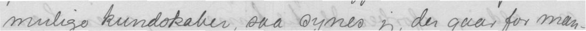mulige kundskaber, saa synes jeg, der gaar for man-
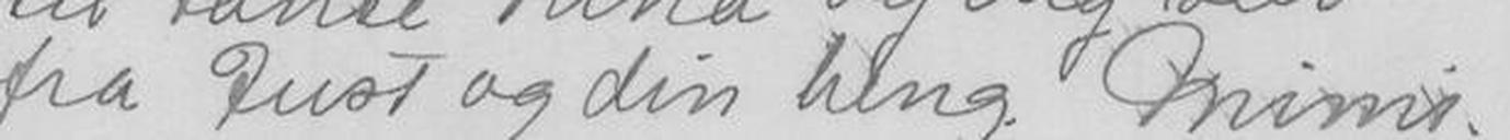fra Just og din heng. Mimi
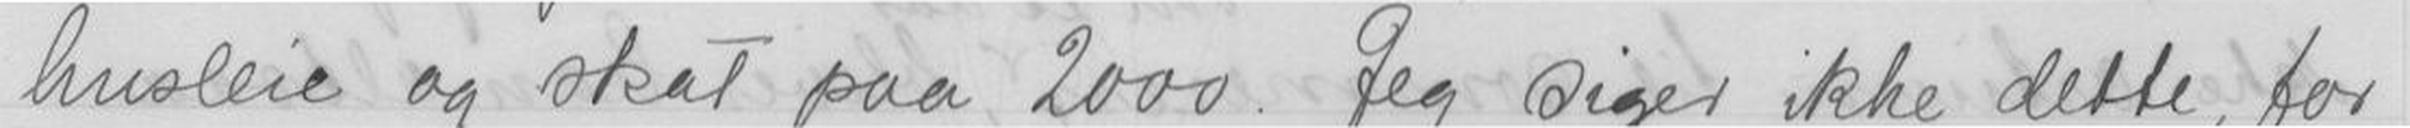husleie og skat paa 2000. Jeg siger ikke dette, for
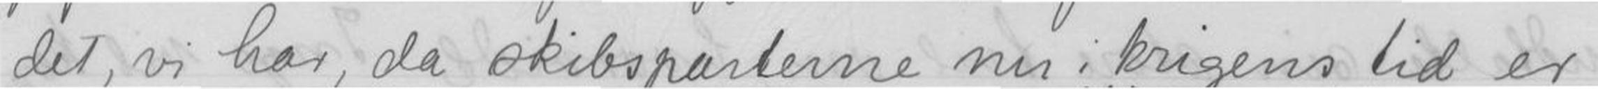det, vi har, da skibsparterne nu i Krigens tid er
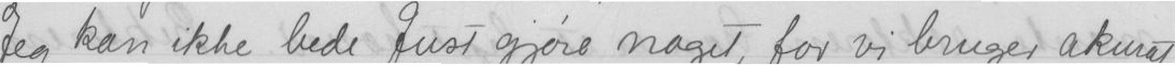Jeg kan ikke bede Just gjøre noget, for vi bruger akurat
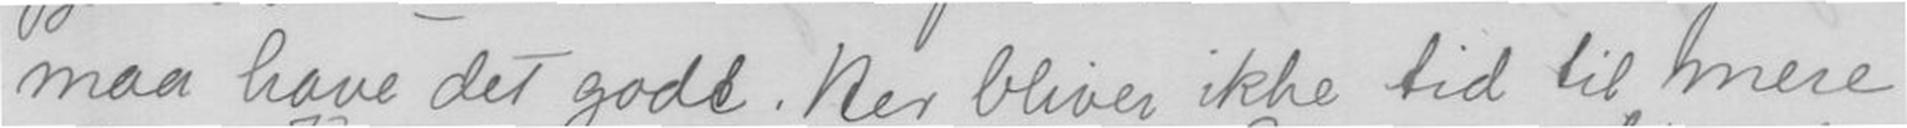maa have det godt. Her bliver ikke tid til mere
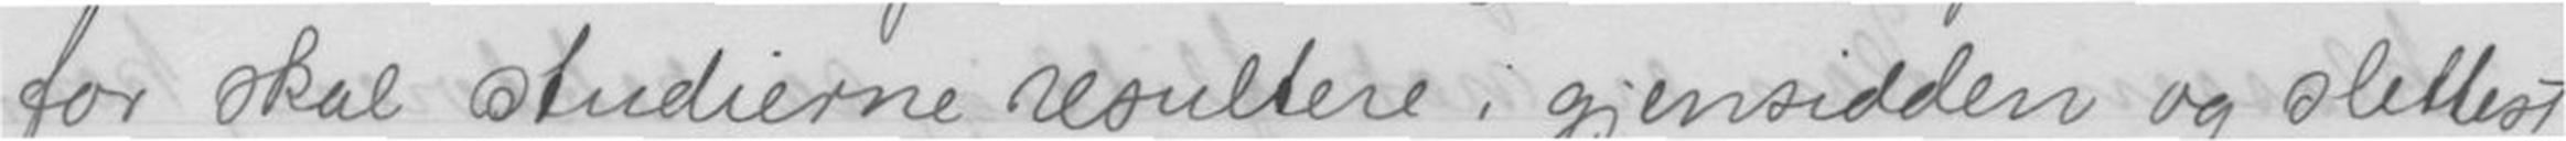for skal studierne resultere i gjensidden og slettest
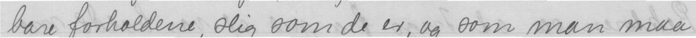bare forholdene, slig som de er, og som man maa
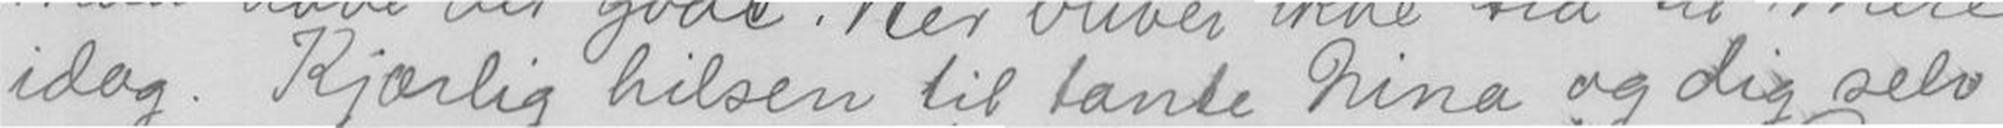idag. Kjærlig hilsen til tante Nina og dig selv
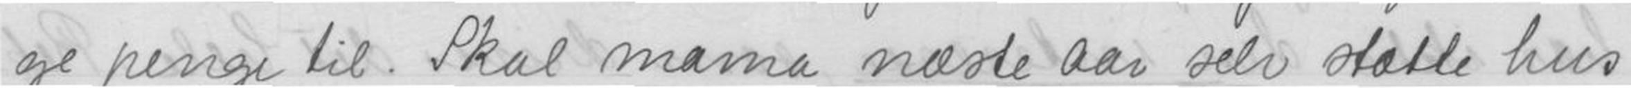ge penge til. Skal mama næste aar selv stætte hus
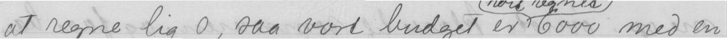at regne lig 0, saa vort budget er 6000 med en
